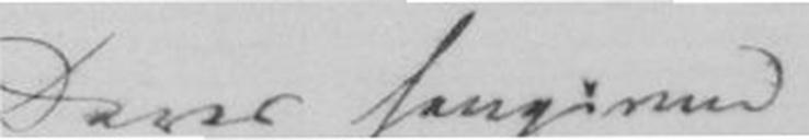Deres hengivne
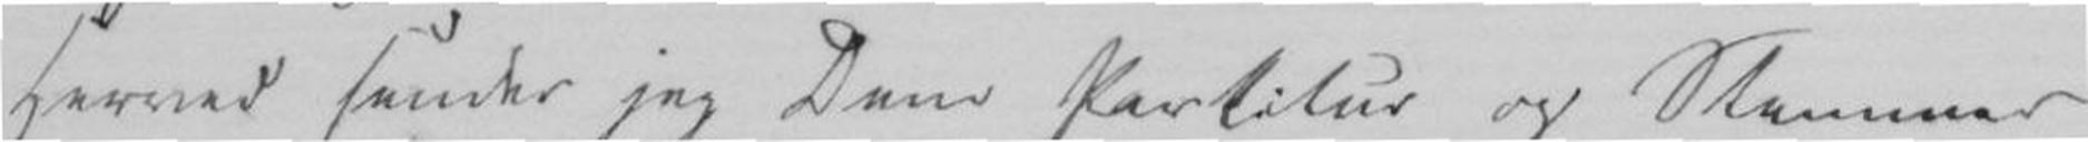Herved sender jeg Dem Patitur og Stemmer
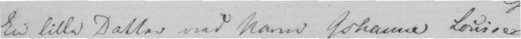En lille Datter ved Navn Johanne Louice
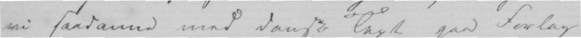vi saadanne med dansk Taxt paa Forlag,
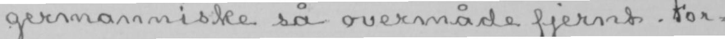germanniske så overmåde fjernt. For-
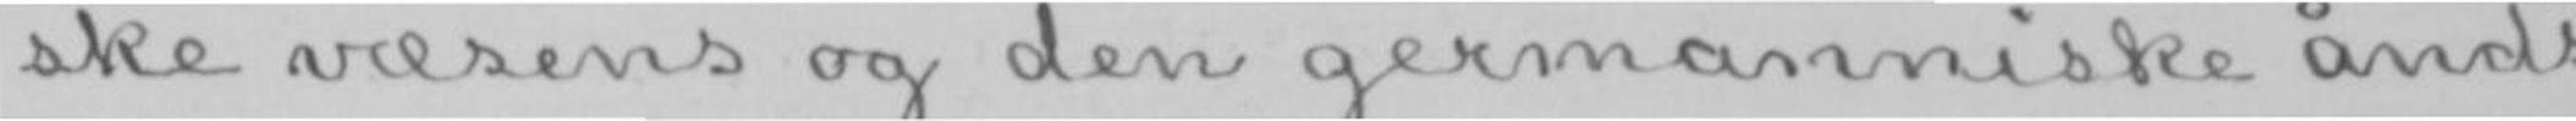ske væsens og den germanniske ånds
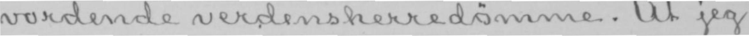vordende verdensherredømme. At jeg
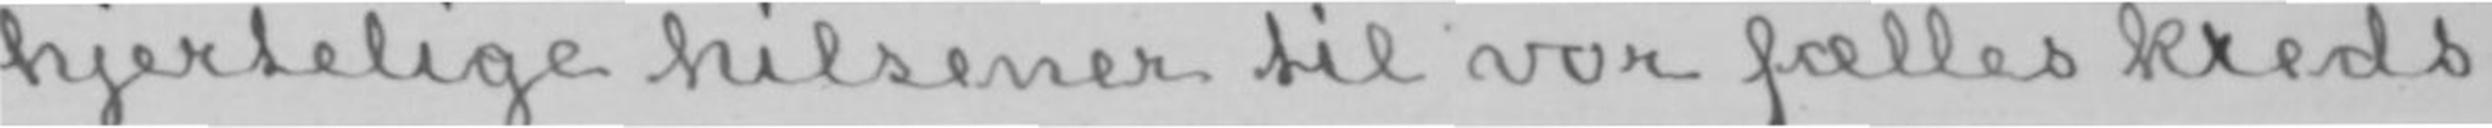hjertelige hilsener til vor fælles kreds
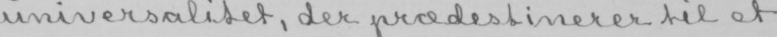universalitet, der prædestinerer til et
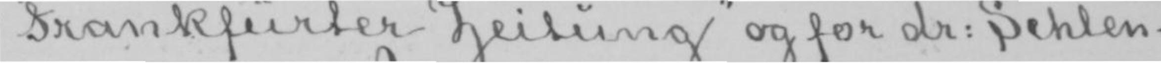«Frankfurter Zeitung» og for dr: Schlen
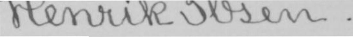Henrik Ibsen.
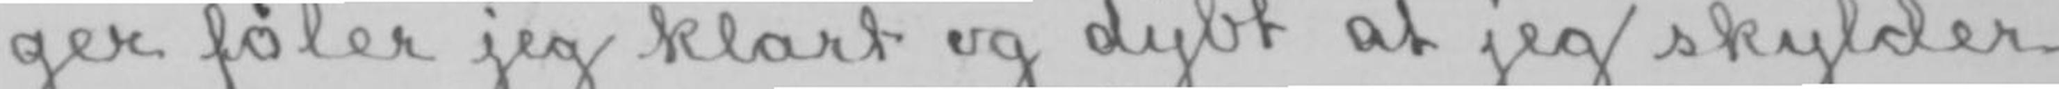ger føler jeg klart og dybt at jeg skylder
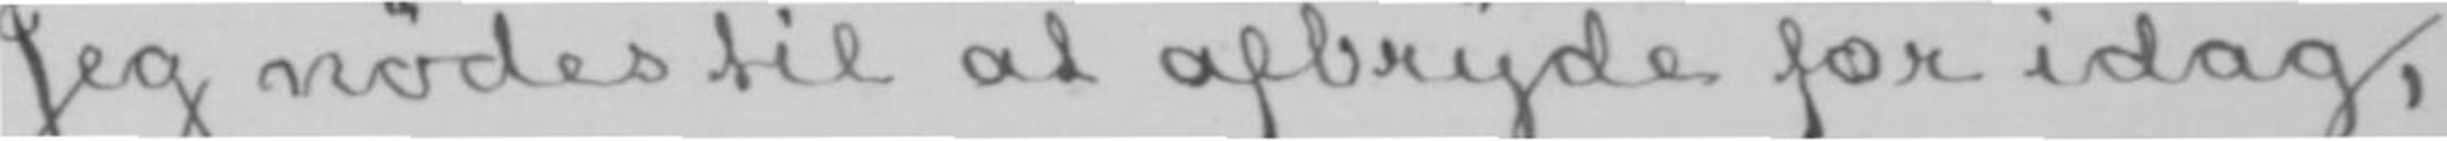Jeg nødes til at afbryde for idag,
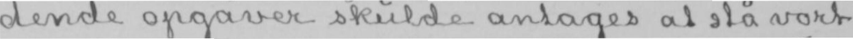dende opgaver skulde antages at stå vort
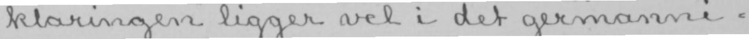klaringen ligger vel i det germanni-
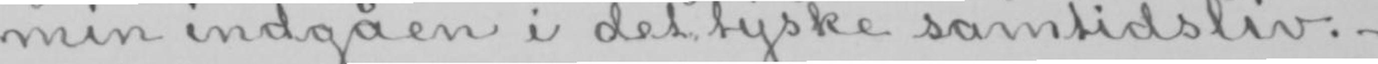min indgåen i det tyske samtidsliv.-
